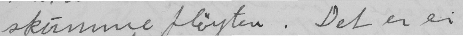skumme flöyten. Det er ei
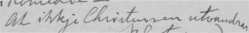At ikkje Christensen utvandrar
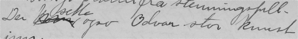Der sette ogso Odvar stor knust
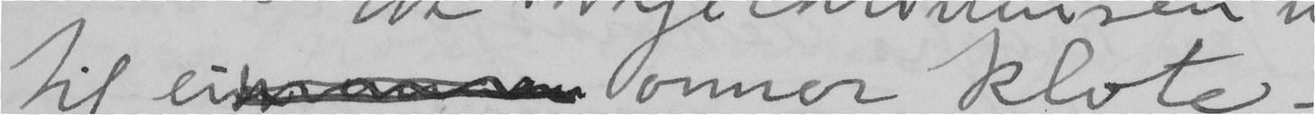til  ei onnen klote –
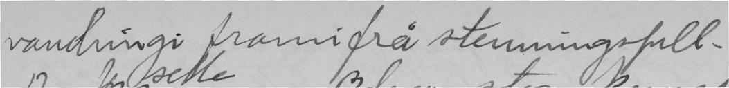vandringi framifrå stemningsfull.
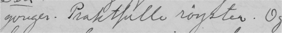gonger. Praktfulle röyster. Og
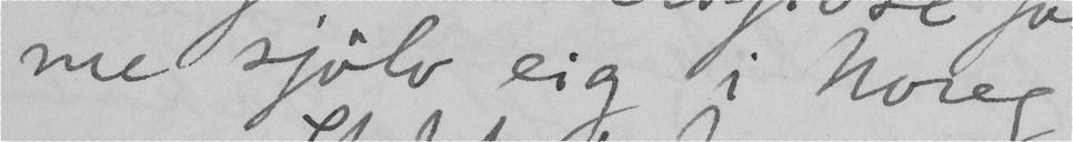me sjölv eig i Noreg –
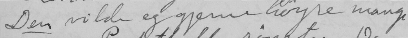Den vilde eg gjerne höyre mange
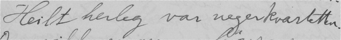Heilt herleg var negerkvartetten.
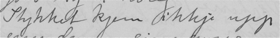Stykket kjem ikkje upp
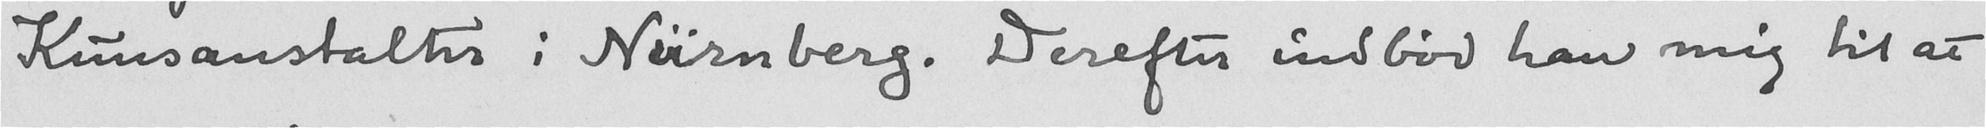Kunsanstalter i Nürnberg. Derefter indbød han mig til at
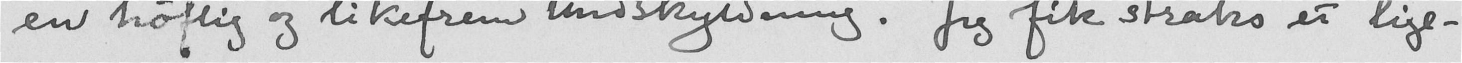en høflig og likefrem undskyldning. Jeg fik straks et lige-
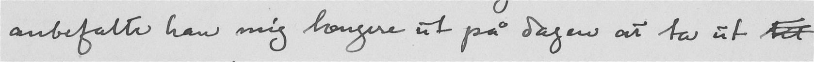anbefalte han mig længere ut på dagen at ta ut til
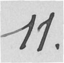11.
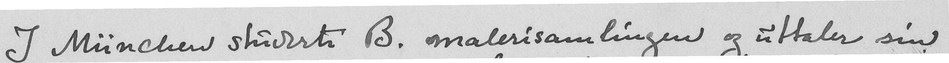I München studerte B. malerisamlingen og uttaler sin
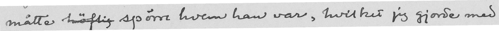måtte høflig spørre hvem han var, hvilket jeg gjorde med
